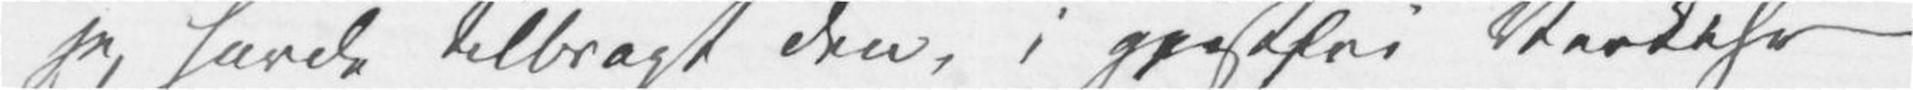jeg havde tilbragt den, i gjestfri Verkehr
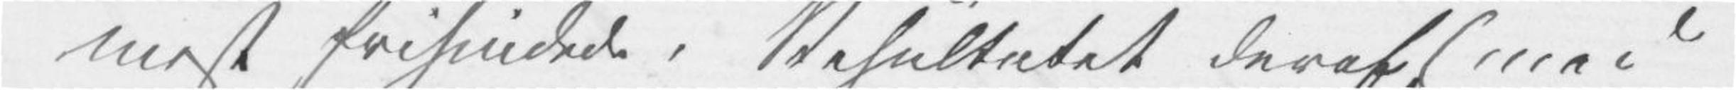mest frisindede. Resultatet deraf (med
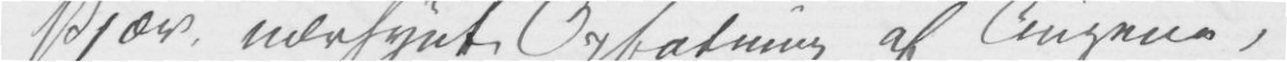skjæv, nærsynt Opfatning af Tingene,
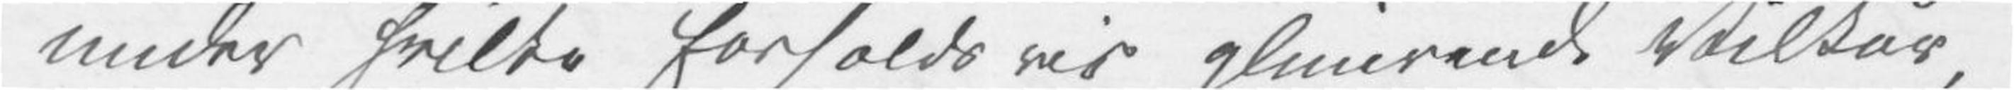under hvilke forholdsvis glimrende Vilkår,
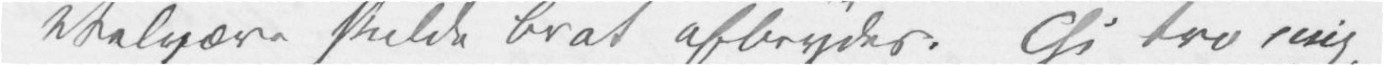Velvære skulde brat afbrydes. Thi tro mig,
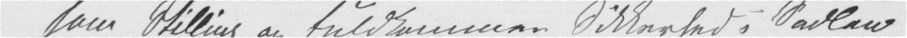som Stilling og fuldkommen Sikkerhed i Sadlen
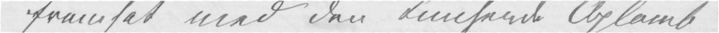fremsat med den knusende Aplomb
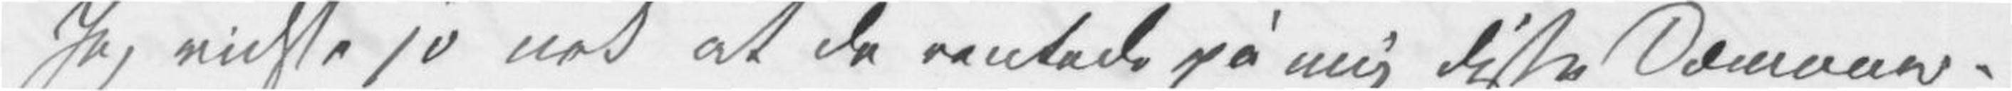Jeg vidste jo nok at de ventede på mig disse Dæmoner.
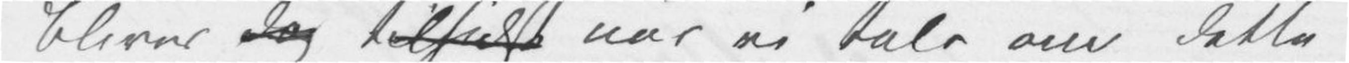bliver dog tilsidst når vi tale om dette
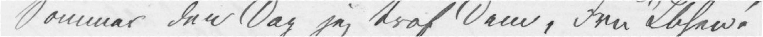Sommer den Dag jeg traf Dem, Fru Ibsen!
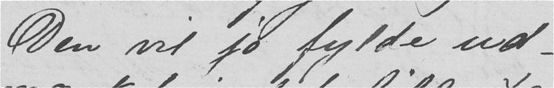Den vil jo fylde ud-
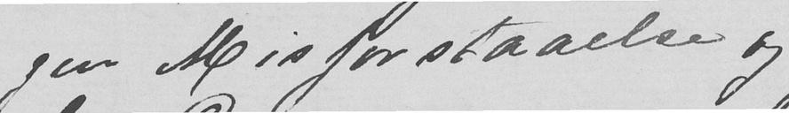gen Misforstaaelse og
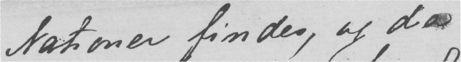Nationer findes, og da
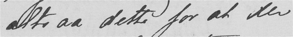altsaa dette for at der
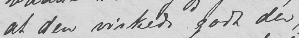at den virkede godt der,
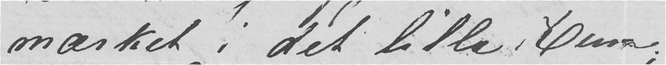mærket i det lille Rum;
